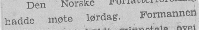hadde møte lørdag. Formannen
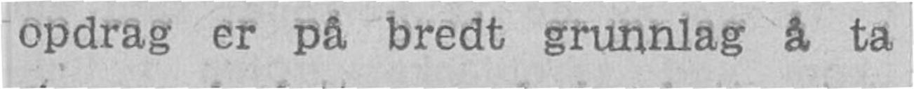opdrag er på bredt grunnlag å ta
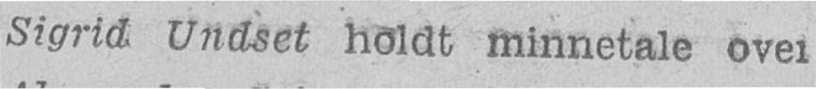Sigrid Undset holdt minnetale over
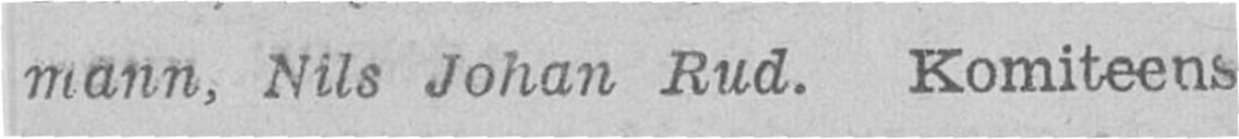mann, Nils Johan Rud. Komiteens
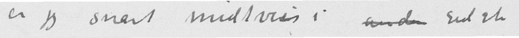er jeg snart midtveis i and sidste
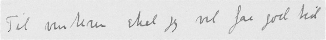Til vinteren skal jeg vel faa god tid
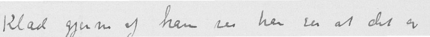Klæd gjerne af ham saa han ser at det er
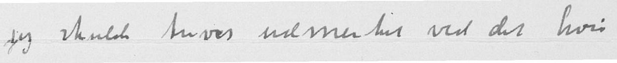jeg skulde trives udmerket ved det hvis
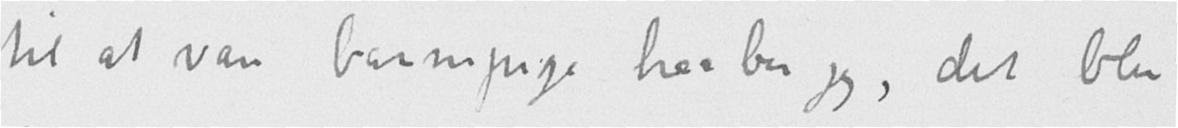til at være barnepige haaber jeg, det blir
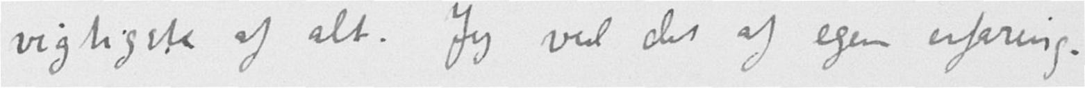vigtigste af alt. Jeg ved det af egen erfaring.
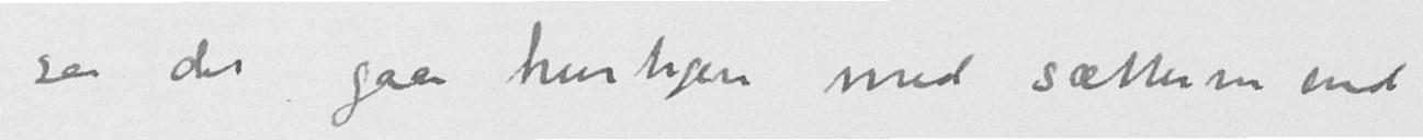saa det gaar hurtigere med sætterne end
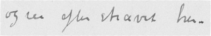ogsaa efter strævet her.
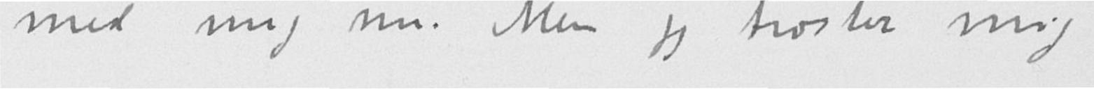med mig nu. Men jeg trøster mig
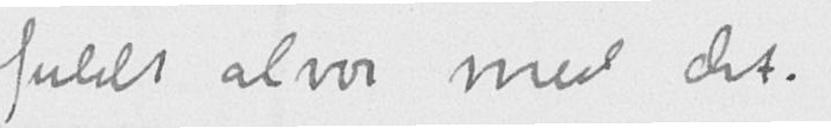fuldt alvor med det.
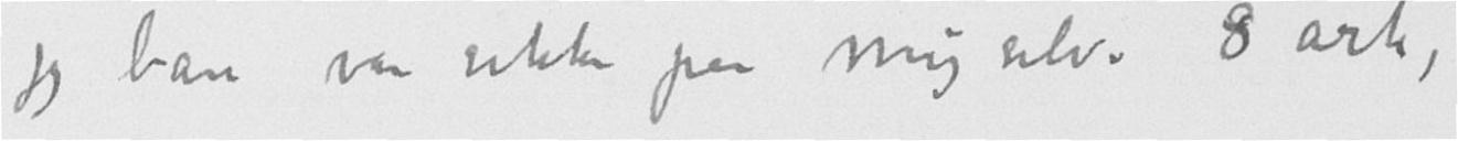jeg bare var sikker paa mig selv. 8 ark,
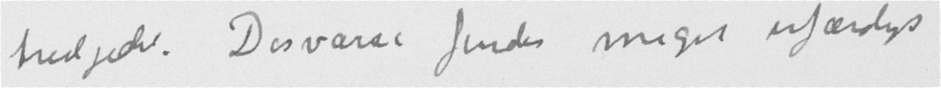tredjedel. Desværre findes meget ufærdigt
